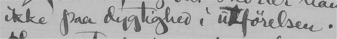ikke paa dygtighed i utførelsen.
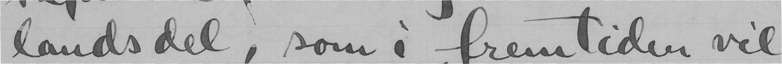landsdel, som i fremtiden vil
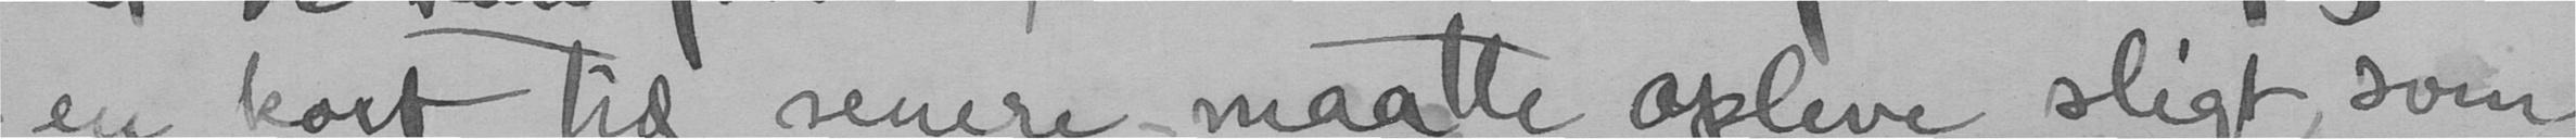- en kort tid senere - maatte opleve sligt som
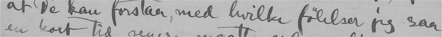at De kan forstaa, med hvilke følelser jeg saa
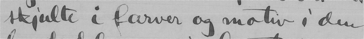skjulte i farver og motiv i den
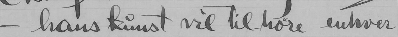- hans kunst vil tilhøre enhver
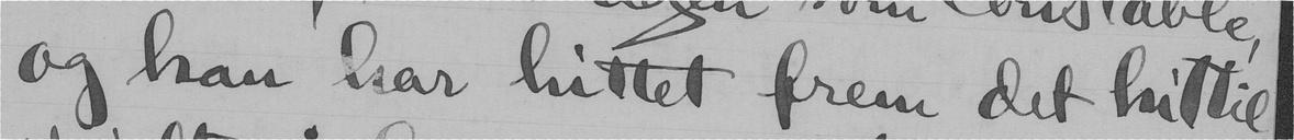og han har hittet frem det hittil
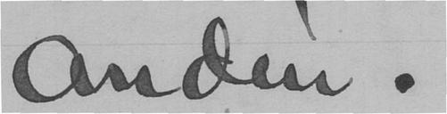anden.
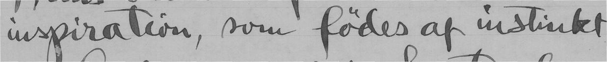inspiration, som fødes af instinkt
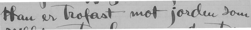Han er trofast mot jorden som
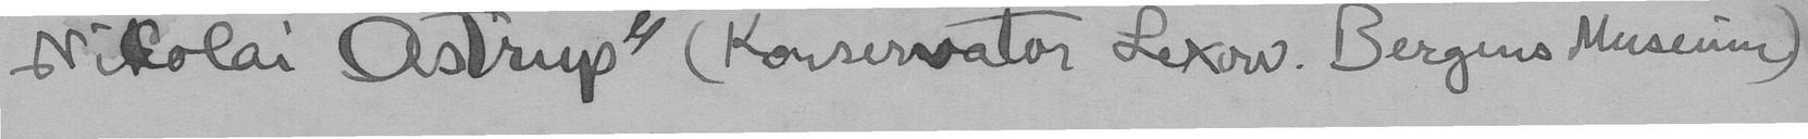Nicolai Astrup" (Konservator Lexow. Bergens Museum)
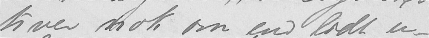tiver nok om end lidt u-
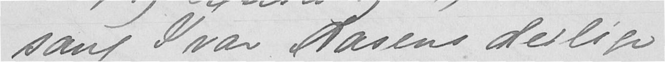sang Ivar Aasens deilige
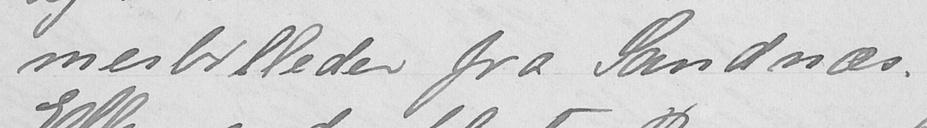merbilleder fra Sandnæs.
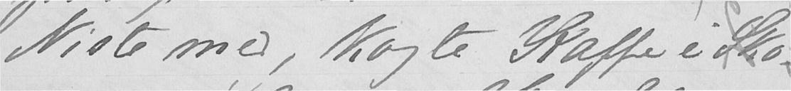Niste med, kogte Kaffe i Sko-
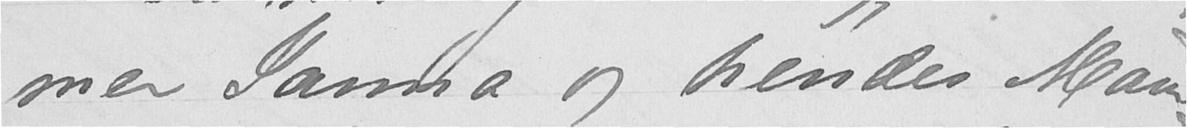mer Janna og hendes Man
